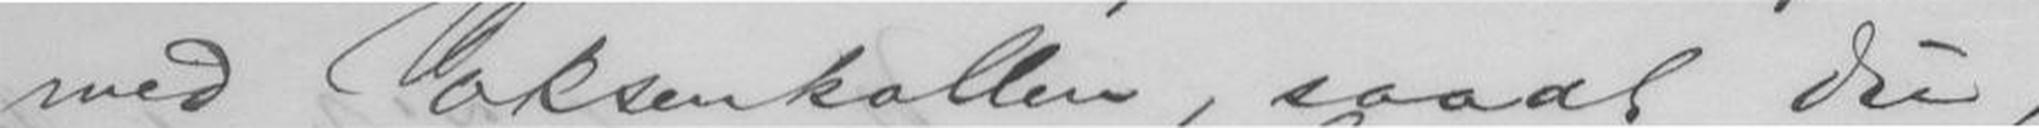med Voksenkollen, saat Du,
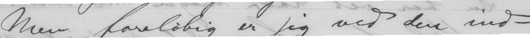Men foreløbig er jeg ved den ind-
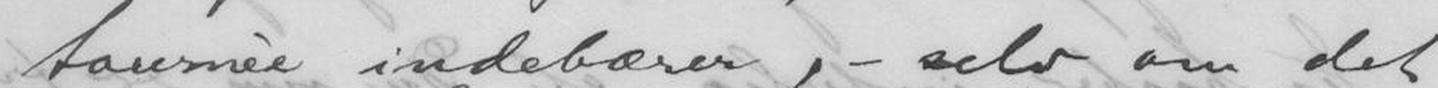tournèe indebærer, - selv om det
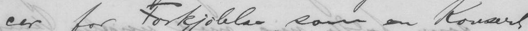cer for Forkjølelse, som en Konsert
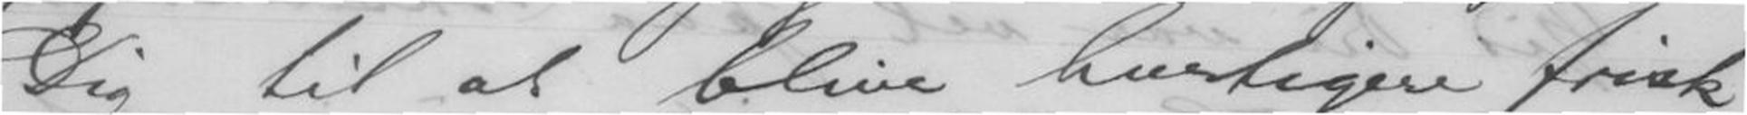Dig til at blive hurtigere frisk,
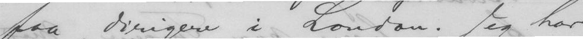faa dirigere i London. Jeg har
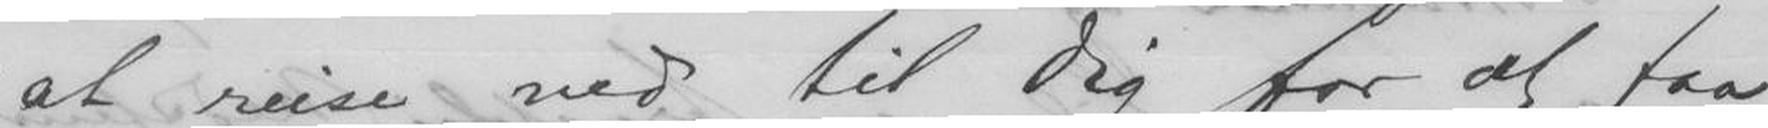at reise ned til Dig for at faa
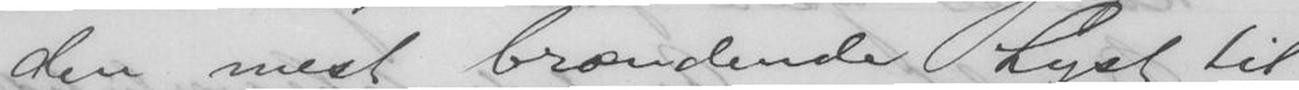den mest brændende Lyst til
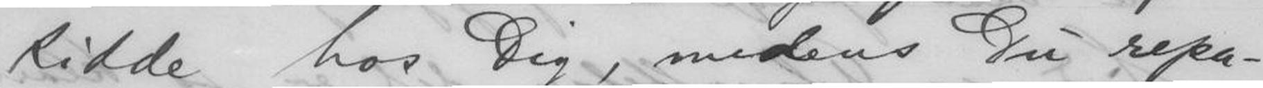sidde hos Dig, medens Du repa-
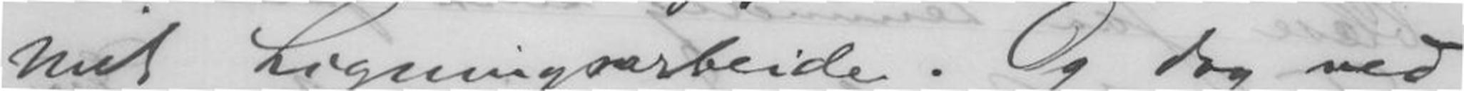mit Ligningsarbeide. Og dog ved
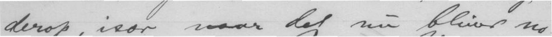derop, især naar det nu bliver no-
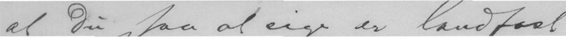at Du saa at sige er landfast
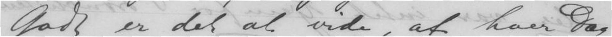Godt er det at vide, at hver Dag
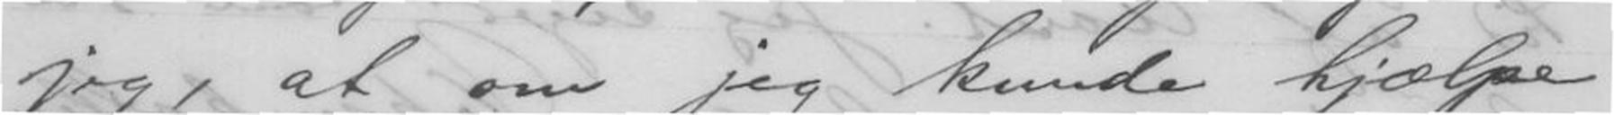jeg, at om jeg kunde hjælpe
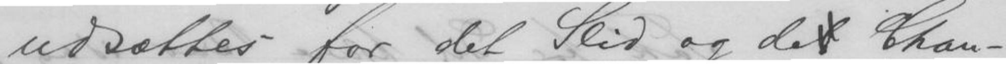udsættes for det Slid og det Chan-
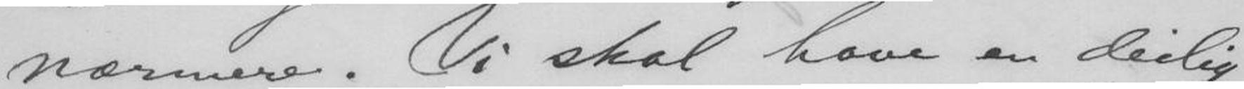nærmere. Vi skal have en deilig
